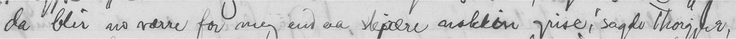da blir vno værre for mig end aa skjære nokkin grise," sagde Thorgjer,
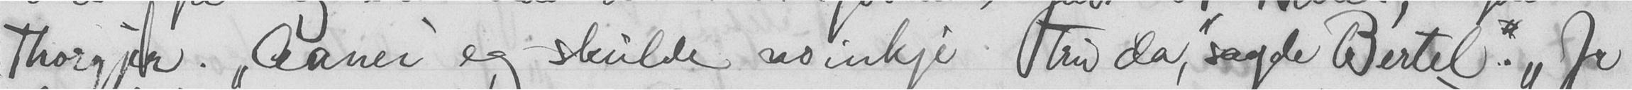Thorgjer. "Aanei eg skulde no inkje tru da," sagde Bertel. "Ja
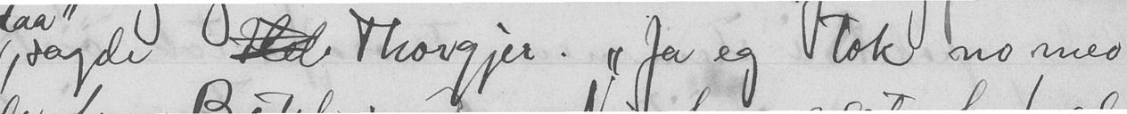, sagde  Thorgjer. "Ja eg tok no med
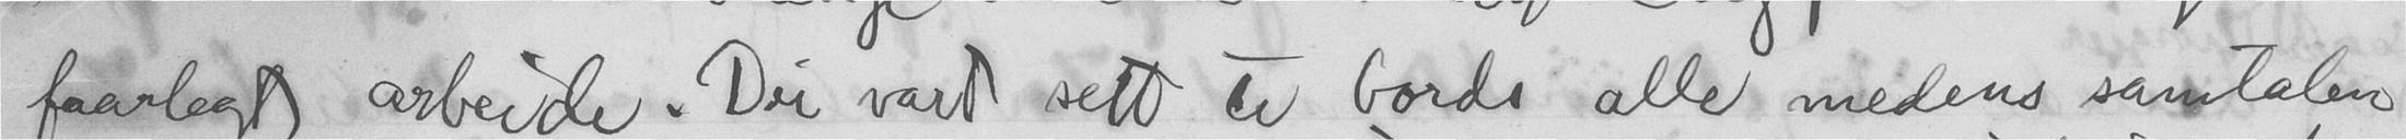faarlegt arbeide. Dei vart sett te bords alle medens samtalen
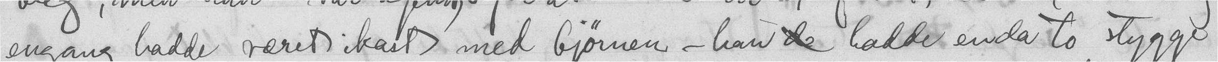engang hadde været ikast med bjørnen - han  hadde enda to stygge
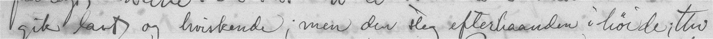gik lavt og hviskende; men den steg efterhaanden i høide, thi
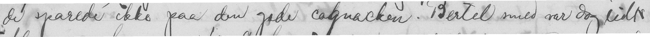de sparede ikke paa den gode cognacken. Bertel smed var dog lidt
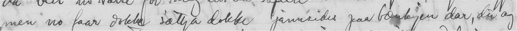"men no faar dokke sættja dokke jamsides paa bænkjen dar, du og
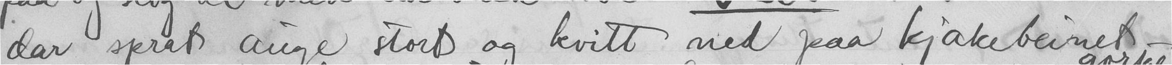dar sprat auge stort og kvitt ned paa kjakebeinet -
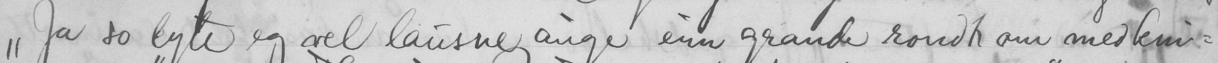"Ja so lyte eg vel lausne auge ein grande rondt om med kvi-
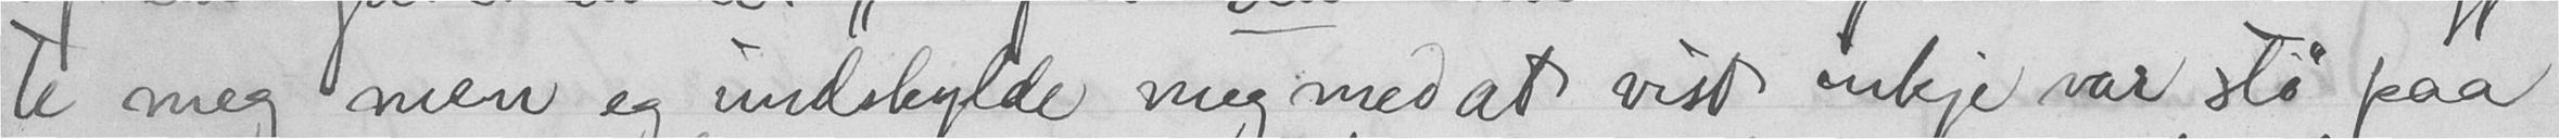te meg men eg undskylde meg med at vist inkje var stø paa
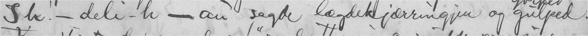Sh! - deli-h - au sagde lægdekjærringjen og gulped.
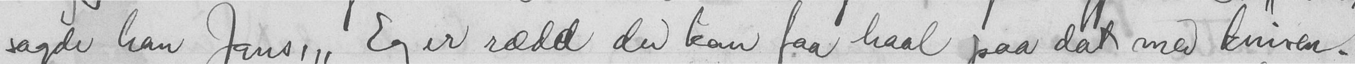sagde han Jans, "Eg er rædd du kan faa haal paa dat med kniven.
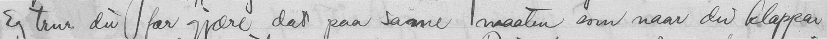Eg trur du far gjære det paa same maaten som naar dur klappar
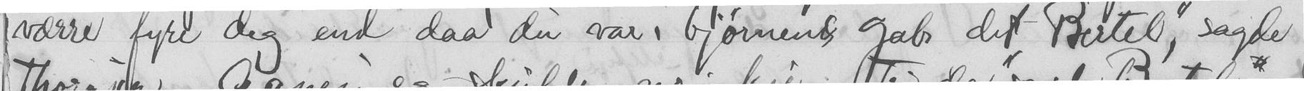værre fyre deg end daa du var i bjørnens gab det Bertel," sagde
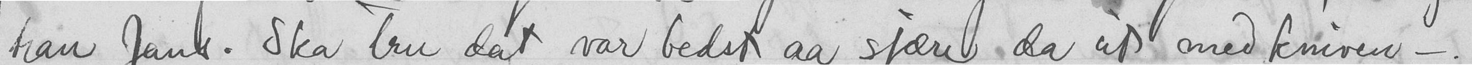han Jans. Ska ha det var bedst aa sjære da ut med kniven -.
